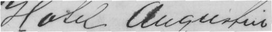Hotel Augustin
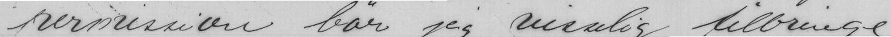permission bør jeg visselig tilbringe
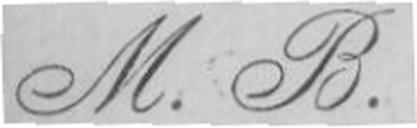M. B.
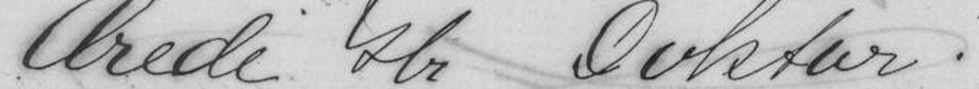Ærede Hr Doktor!
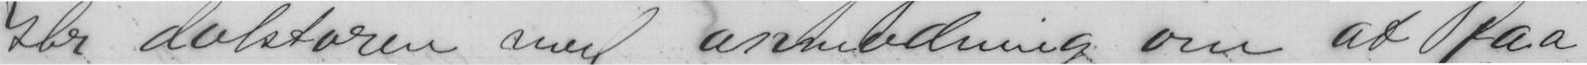Hr Doktoren med anmodning om at faa
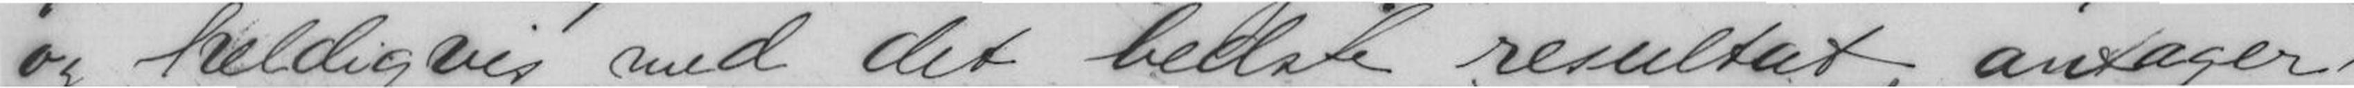og heldigvis med det bedste resultat, antager
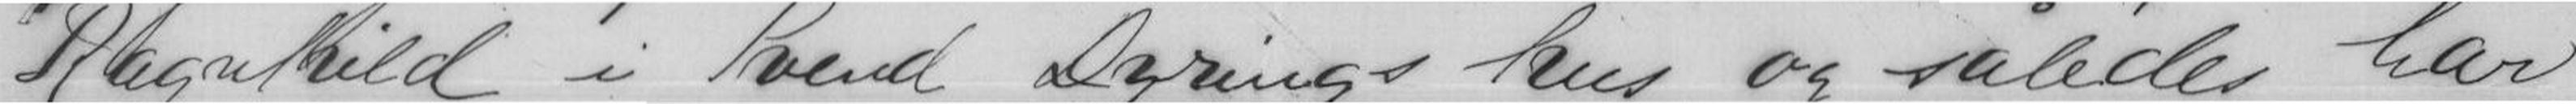Ragnhild i Svend Dyrings hus og således har
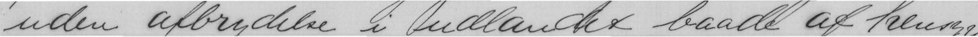uden afbrydelse i udlandet baade af hensyn
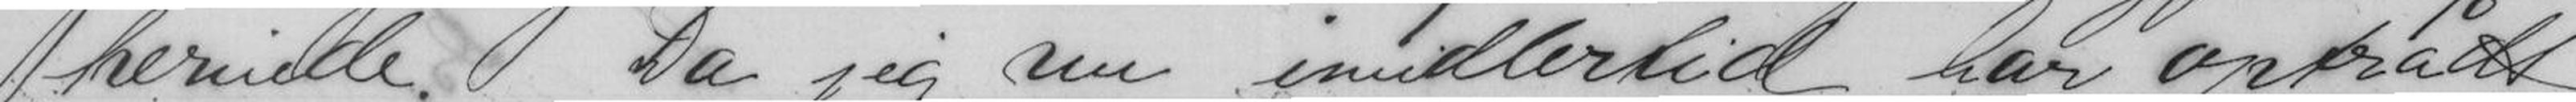herinde. Da jeg nu imidlertid har optrått
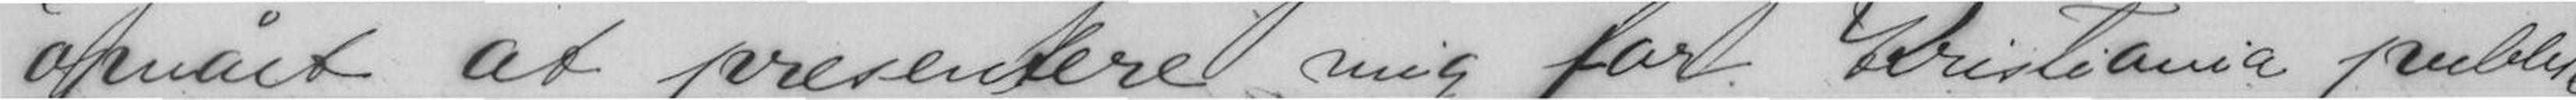opnået at presentere mig for Kristiania publikum
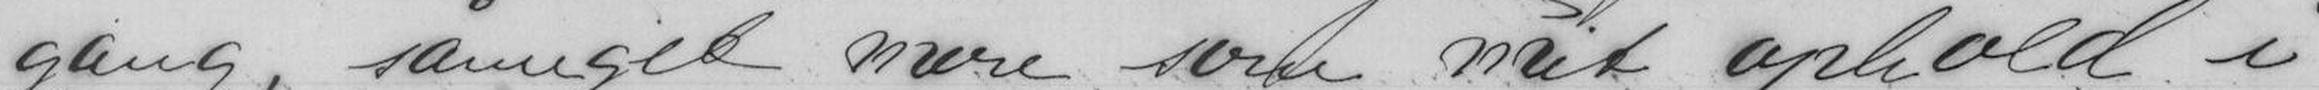gang, såmeget mere som mit ophold i
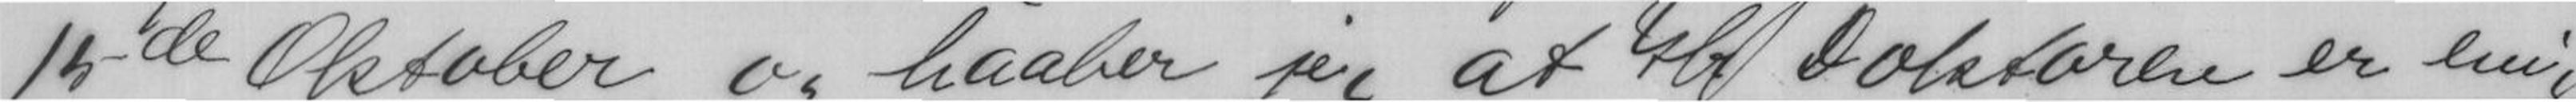15de Oktober og haaber jeg at Hr Doktoren er enig
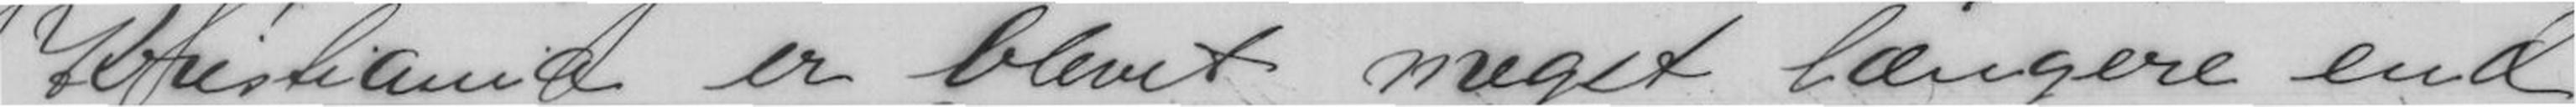Kristiania er blevet meget længre end
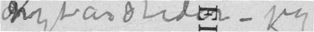nytårstid – jeg
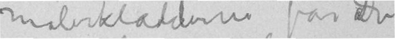malerkladderne får Du
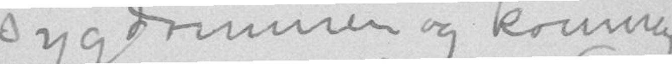sygdommen og kommer
